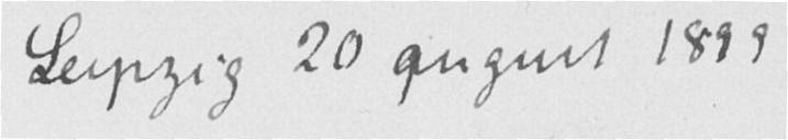Leipzig 26 august 1899
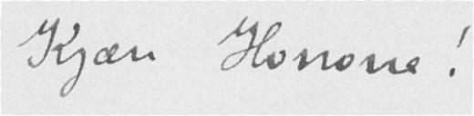Kjære Honoria!
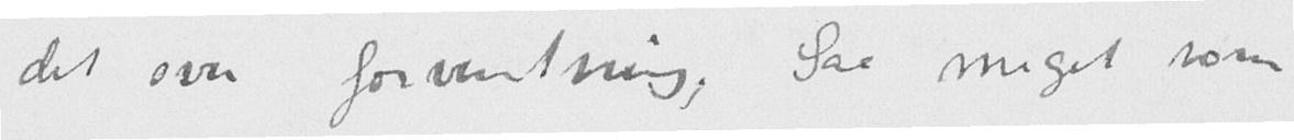det over forventning; Saa meget som
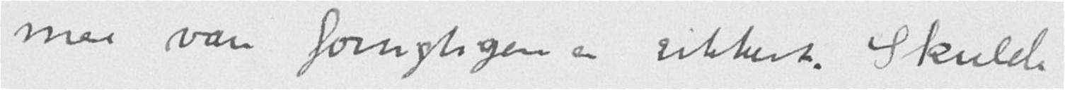maa være forsigtigere er sikkert. Skulde
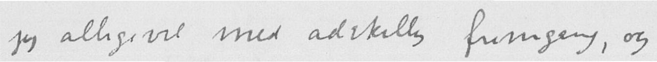jeg alligevel med adskillig fremgang, og
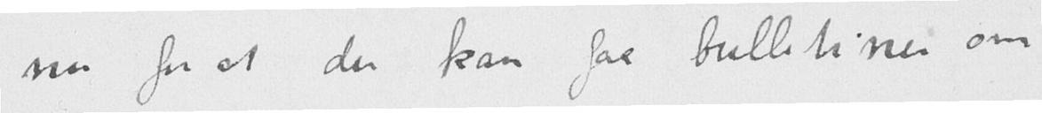nu for at du kan faa bulletiner om
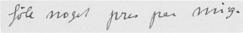føle noget pres paa mig.
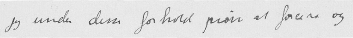jeg under disse forhold prøve at forcere og
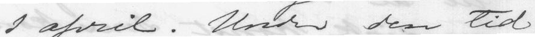1 april. Under den Tid
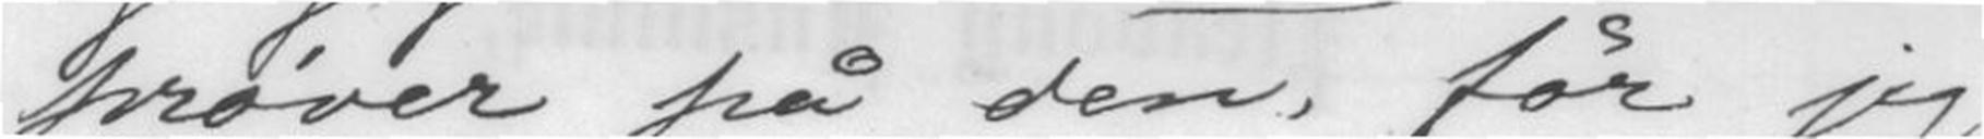prøver på den, får jeg
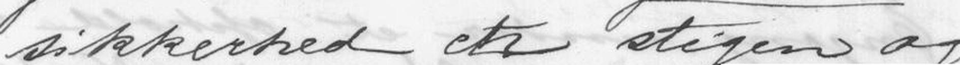sikkerhed en stigen og
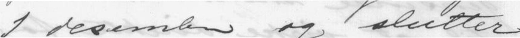1 desember og slutter
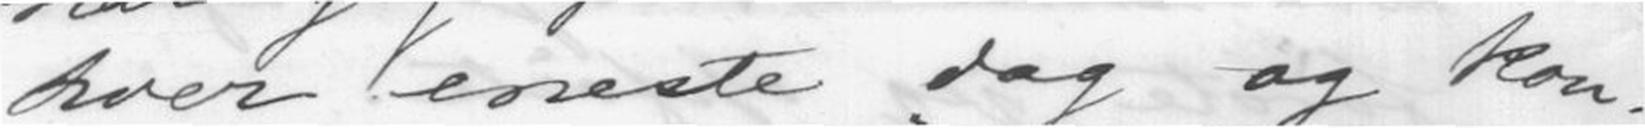hver eneste dag og kon-
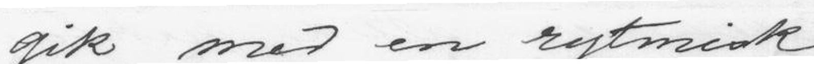gik med en rytmisk
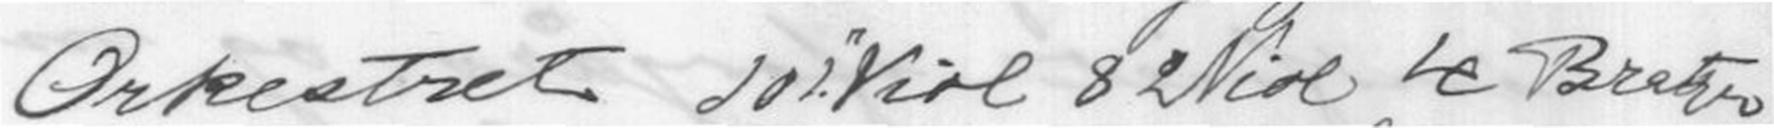Orkesteret 20 1.e Viol 8 2 Viol 4 Bratzer
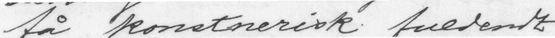få konstnerisk fuldendt
